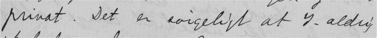privat. Det er sørgeligt at I. aldrig
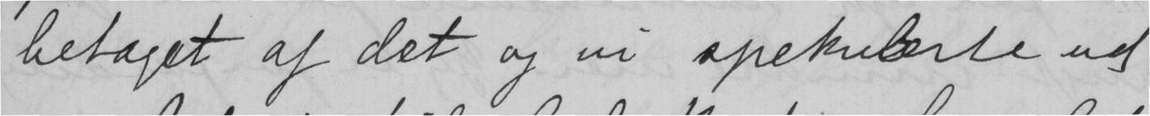betaget af det og vi spekulerte ud
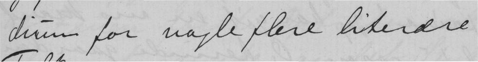dium for nogle flere literære
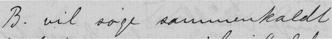B. vil søge sammenkaldt
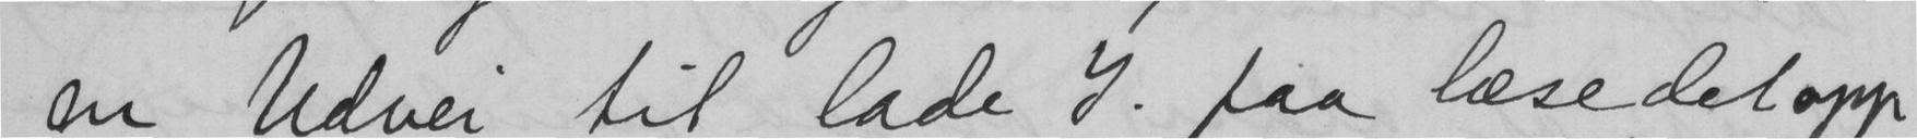en Udvei til lade I. faa læse det opp
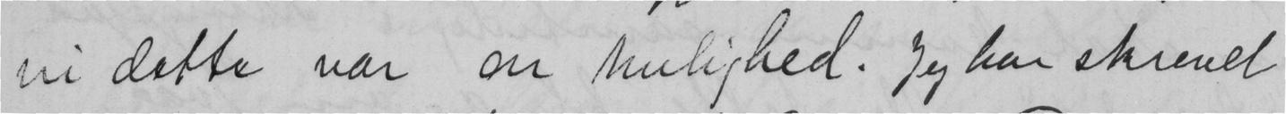e dette var en Mulighed. Jeg har skrevet
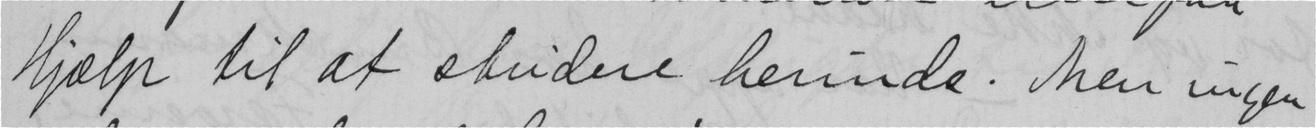Hjælp til at studere herinde. Men ingen
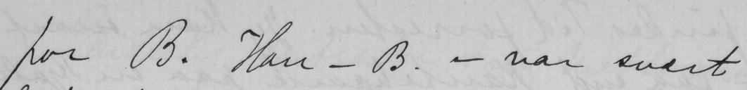for B. Han - B. - var svært
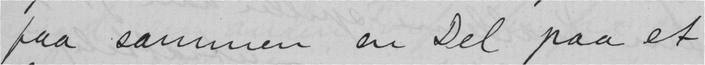faa sammen en Del paa et
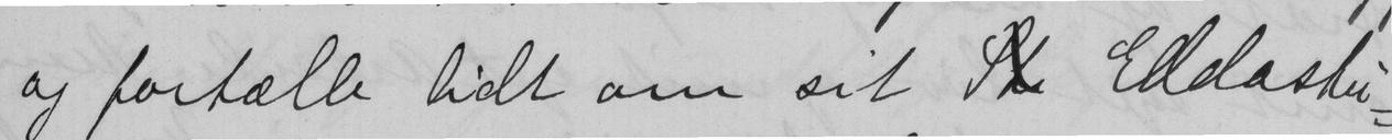og fortælle lidt om sit  Eddastu-
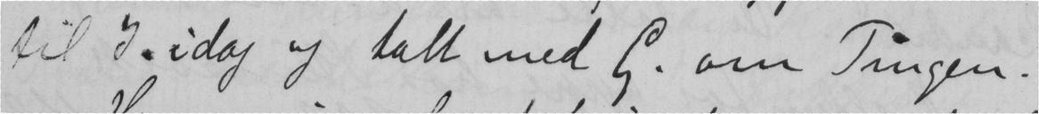til I. idag og talt med G. om Tingen.
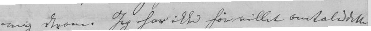mig derom. Jeg har ikke før villet omtale dette
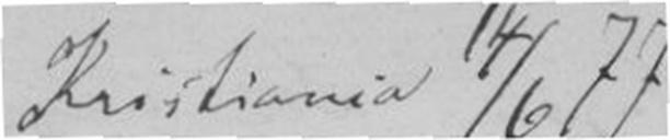Kristiania 14/6 77
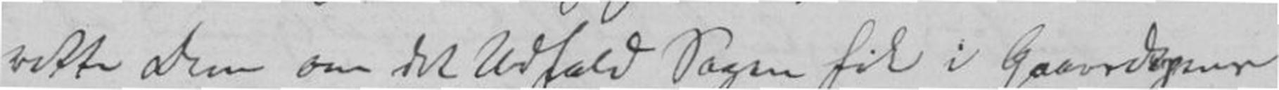rette Dem om Udfald Sagen fik i Gaarsdagens
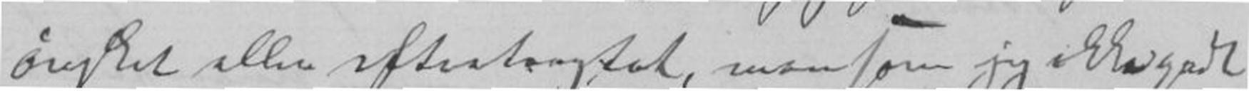ønsket eller efterlengtet, men som jeg ikke godt
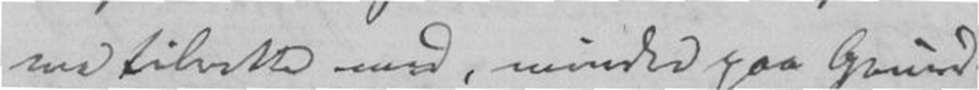me tilrette med, mindre paa Grund
